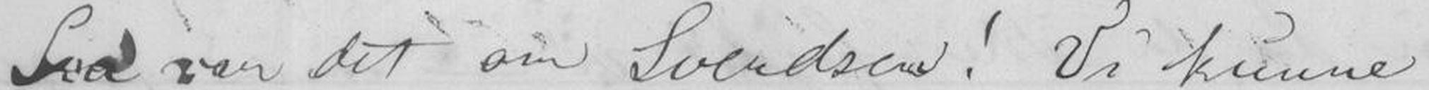Saa var det om Svendsen! Vi kunne
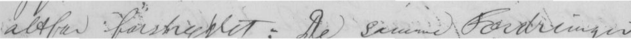altfor forskrækket: De samme Fordringer
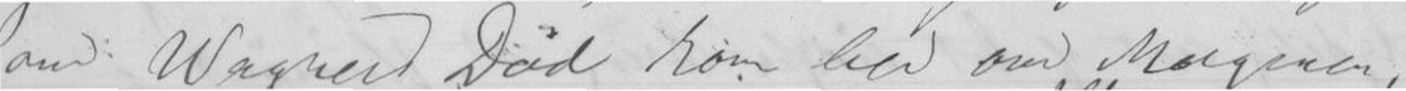om Wagners Død kom her om Morgenen,
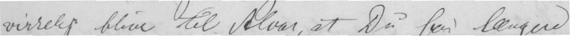virkelig blive til Alvor, at Du for længere
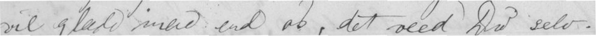vil glæde mere end os, det veed Du selv.
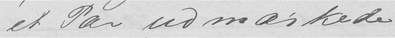et Par udmærkede
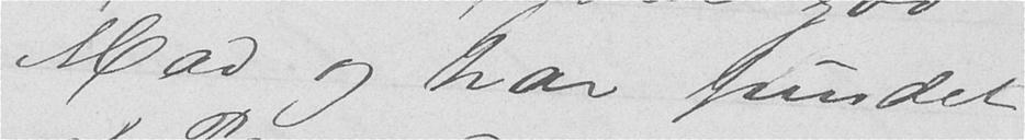Mad og har fundet
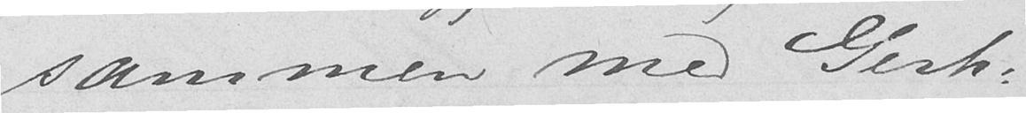sammen med Gerh:
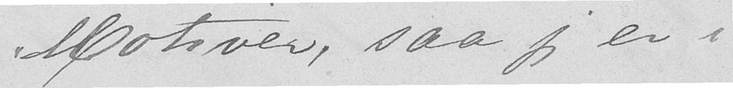Motiver, saa jeg er i
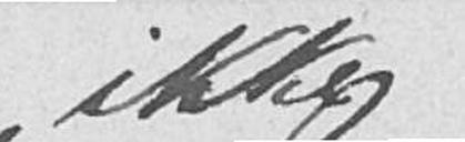ikke
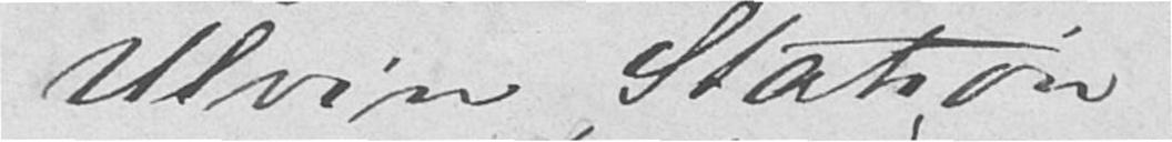Ulvin Station
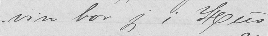vin bor jeg i Hus
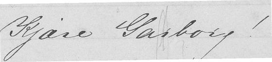Kjære Garborg!
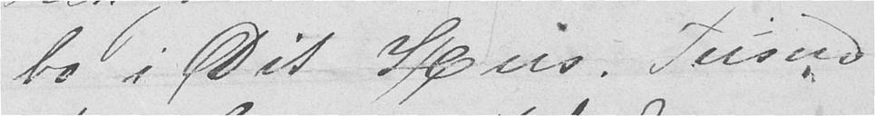bo i Dit Hus. Tusind
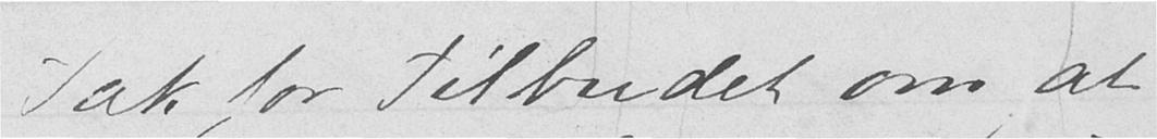Tak for Tilbudet om at
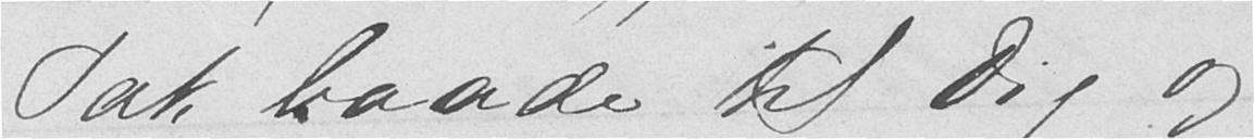Tak baade til Dig og
